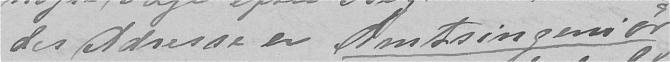des Adresse er Amtsingeniør
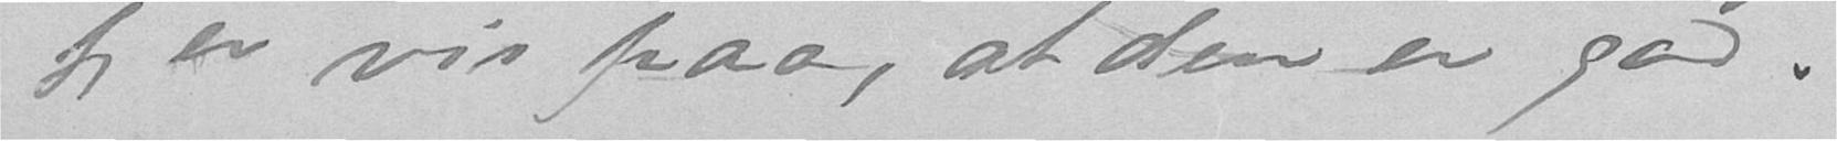jeg er vis paa, at den er god.
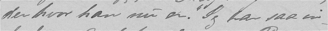der hvor han nu er. Jeg har saa in-
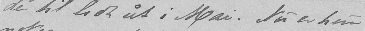der til lidt ut i Mai. Nu er hun
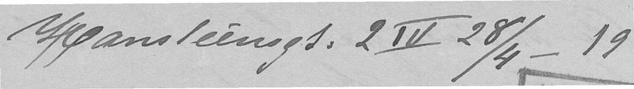Hansteensgt: 2 IV 28/4 - 19
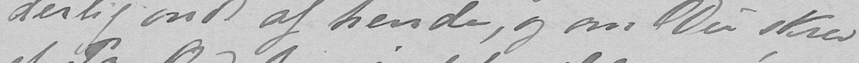derlig ondt af hende, og om Du skrev
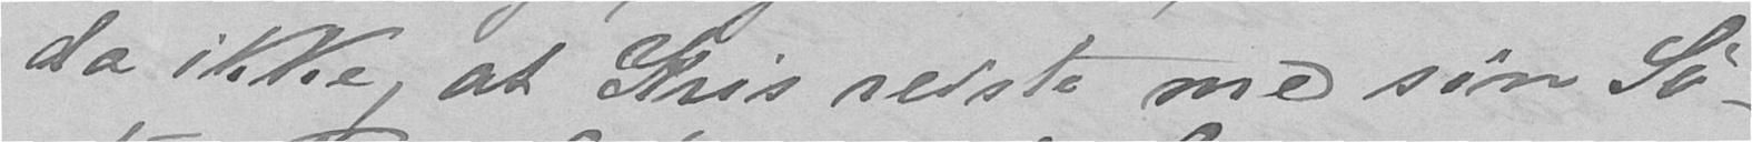da ikke, at Kris reiste med sin Sø-
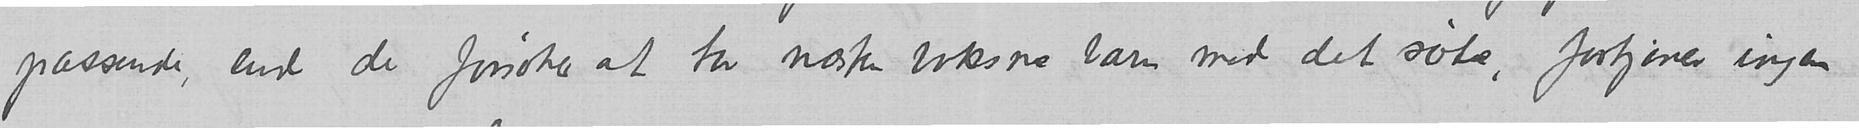passende, end de forsøker at ta næsten voksne barn med det søte, fortjener ingen
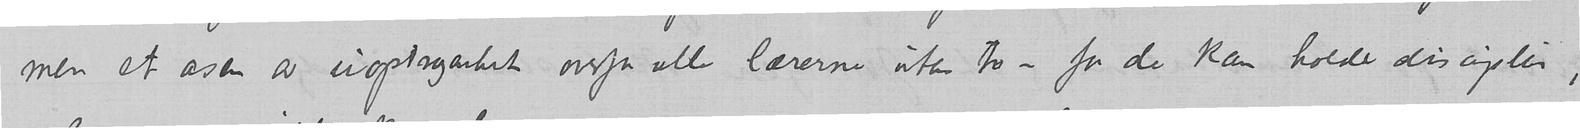men et asen av uopdragenhet overfor alle lærerne uten to – for de kan holde disciplin,
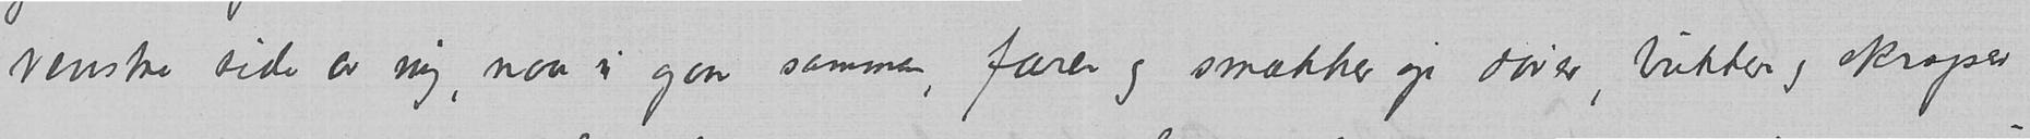venstre side av mig, naar vi gaar sammen, farer og smækker op dører, bukker og skraper
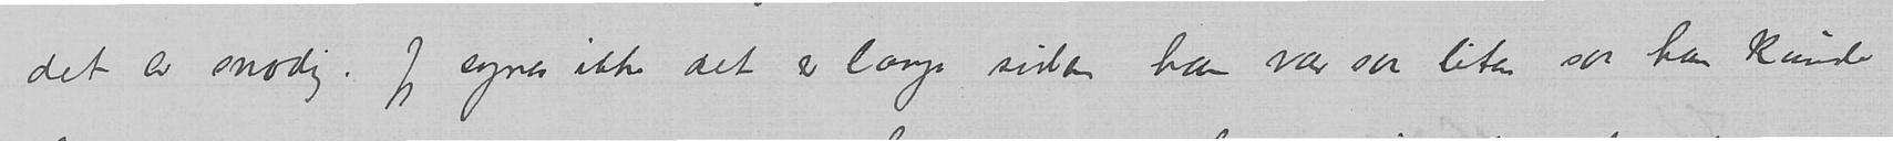det er snodig. Jeg synes ikke det er længe siden han var saa liten saa han kunde
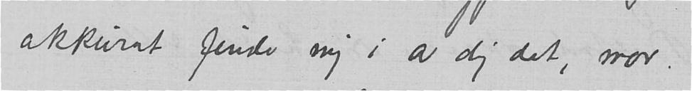akkurat finde mig i av dig det, mor.»
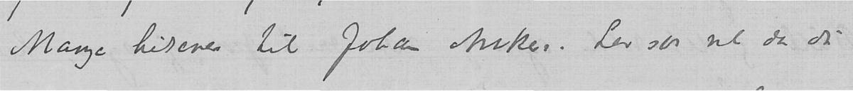Mange hilsener til Johan Anker. Lev saa vel da du
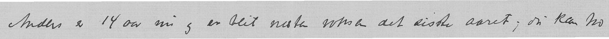Anders er 14 aar nu og er blit næsten voksen det sisste aaret; du kan tro
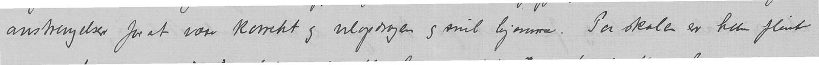anstrengelser for at være korrekt og velopdragen og snil hjemme. 	Paa skolen er han flink
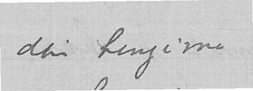din hengivne
